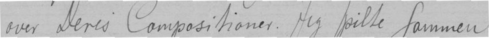over Deres Compositioner. Jeg spilte sammen
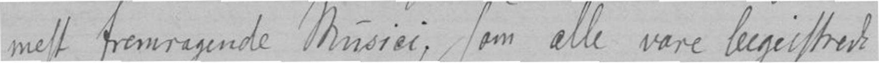mest fremragende Musici, som alle være begeistrede
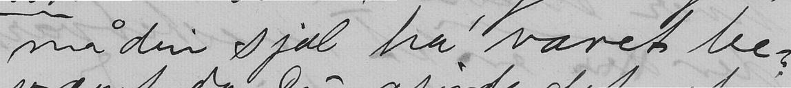må din sjæl ha' været be-
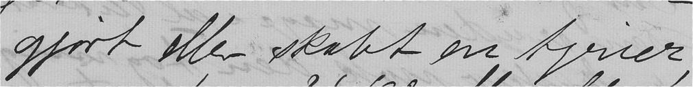gjort eller skabt en tjener,
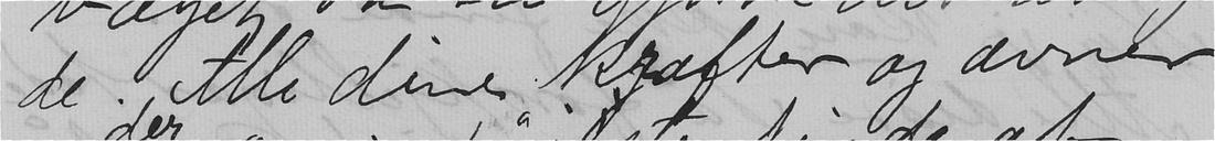de. Alle dine kræfter og ævner
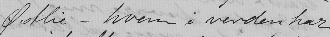Østlie - hvem i verden har
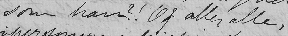som han?! Og alle, alle,
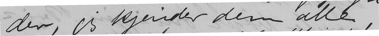der, jeg kjender dem alle,
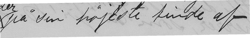på sin højeste tinde af
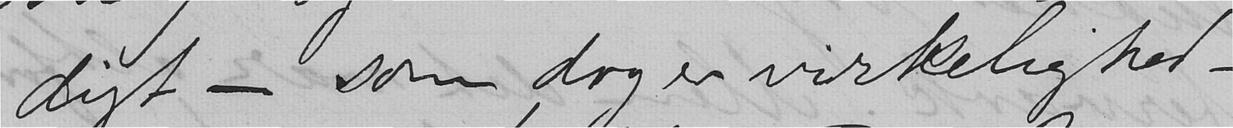digt - som dog er virkelighed -
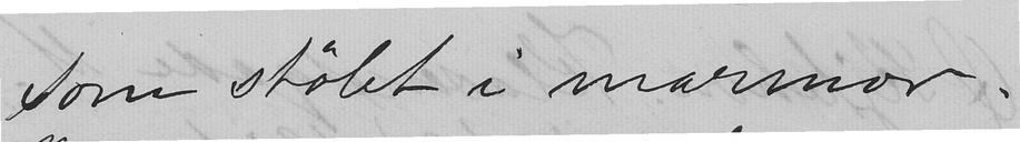som støbt i marmor.
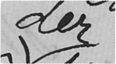der
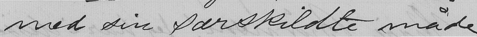med sin særskildte måde
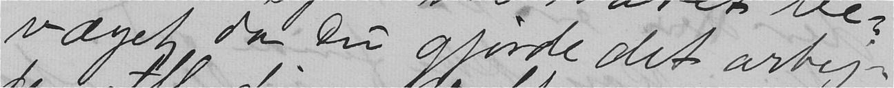væget da Du gjorde det arbej-
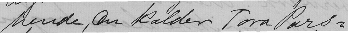hende, Du kalder Tora Pars-
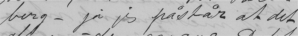berg - ja jeg påstår at det
